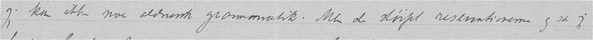jeg kan ikke noe oldnorsk grammatik. Men de sløifet reservationerne og sa jeg
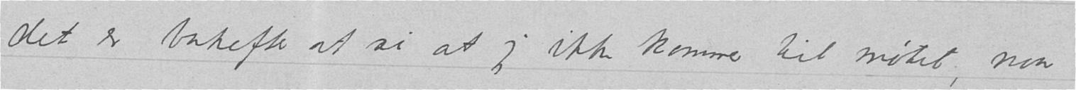det er bakefter at si at jeg ikke kommer til møtet; naar
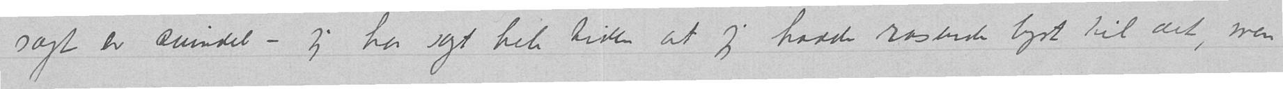sagt er svindel – jeg har sagt hele tiden at jeg hadde rasende lyst til det, men
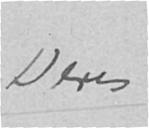Deres
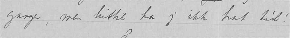ganger, men hittil har jeg ikke hat tid!
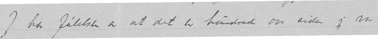Jeg har følelsen av at det er hundrede aar siden jeg var
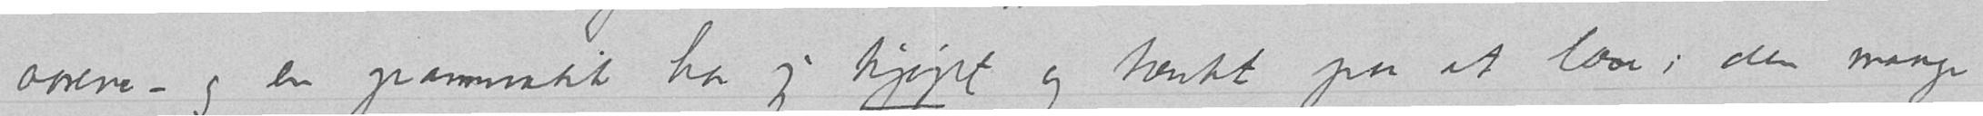aarene – og en grammatik har jeg kjøpt og tænkt paa at læse i den mange
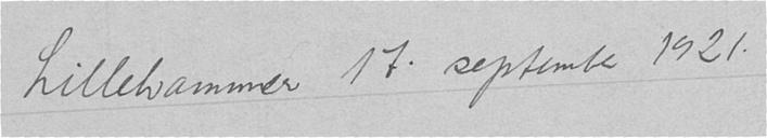Lillehammer 17. september 1921.
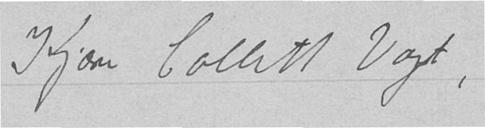Kjære Collett Vogt,
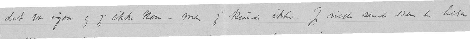det var igaar og jeg ikke kom – men jeg kunde ikke. Jeg vilde sende Dem en hilsen
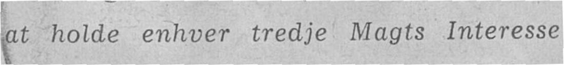at holde enhver tredje Magts Interesse
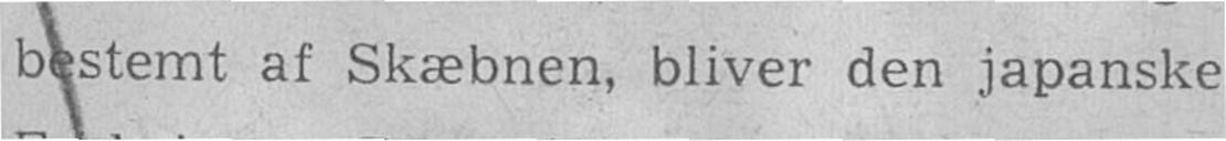bestemt af Skæbnen, bliver den japanske
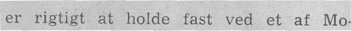er rigtigt at holde fast ved et af Mo-
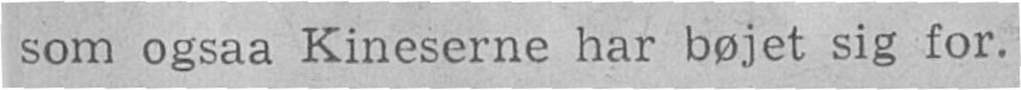som ogsaa Kineserne har bøjet sig for.
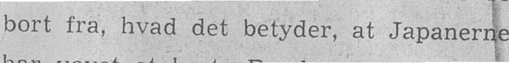bort fra, hvad det betyder, at Japanerne
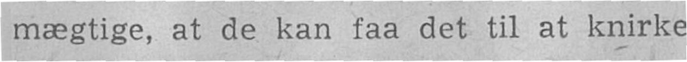mægtige, at de kan faa det til at knirke
